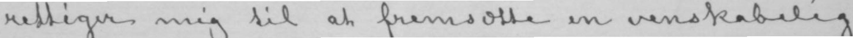rettiger mig til at fremsætte en venskabelig
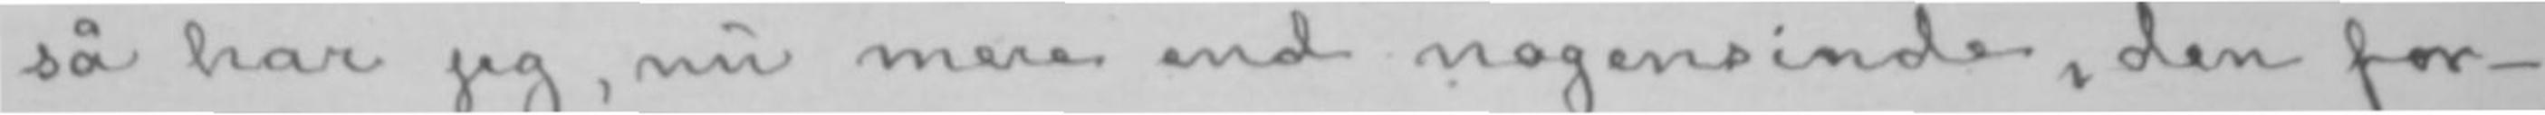så har jeg, nu mere end nogensinde, den for-
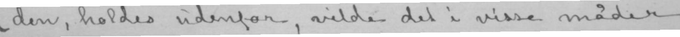den, holdes udenfor, vilde det i visse måder
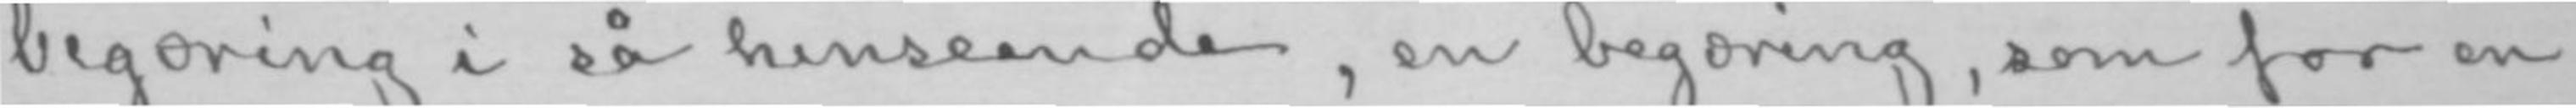begæring i så henseende, en begæring, som for en
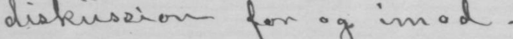diskussion for og imod.
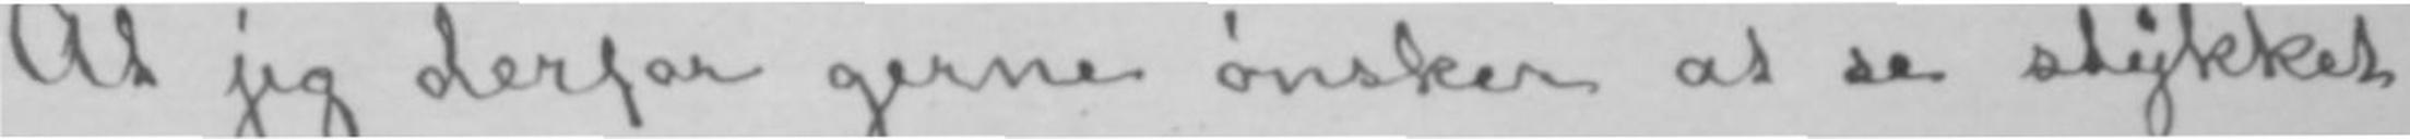At jeg derfor gerne ønsker at se stykket
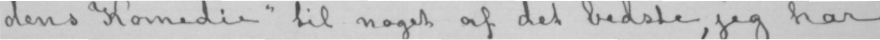dens Komedie» til noget af det bedste, jeg har
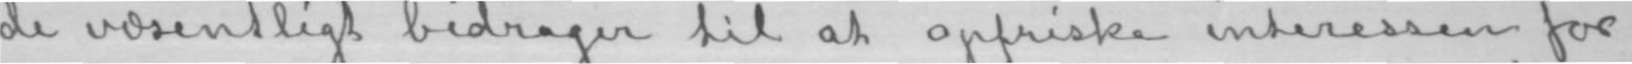de væsentligt bidrager til at opfriske interessen for
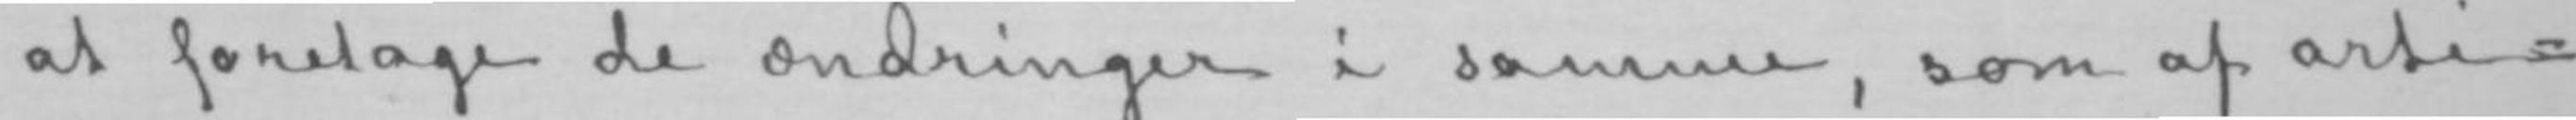at foretage de ændringer i samme, som af arti-
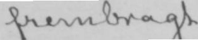frembragt.
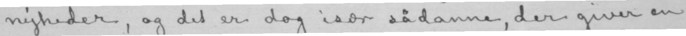nyheder, og det er dog især sådanne, der giver en
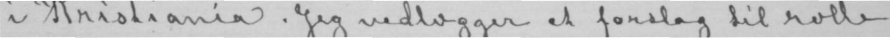i Kristiania. Jeg vedlægger et forslag til rolle-
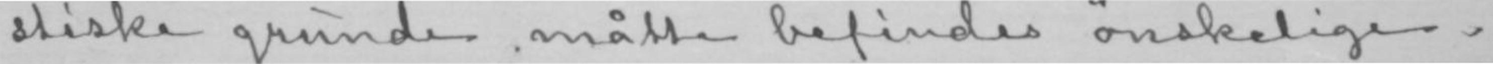stiske grunde måtte befindes ønskelige.
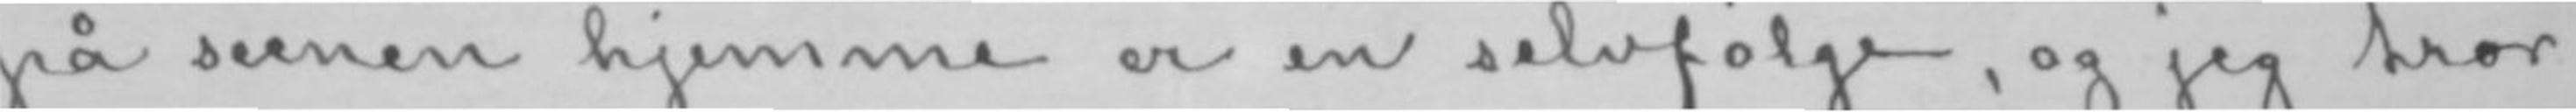på scenen hjemme er en selvfølge, og jeg tror
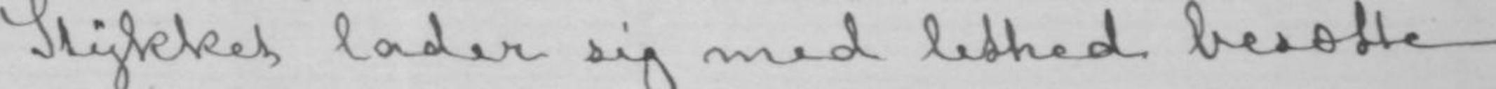Stykket lader sig med lethed besætte
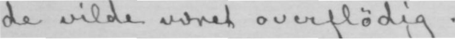de vilde været overflødig.
